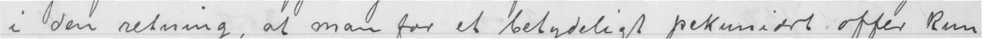i den retning, at man for et betydeligt pekuniært offer kun
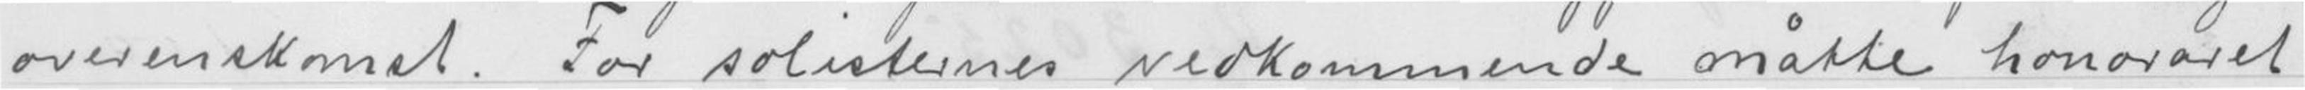overenskomst. For solisternes vedkommende måtte honoraret
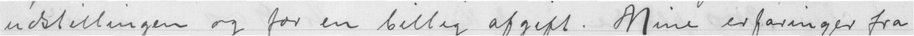udstillingen og for en billig afgift. Mine erfaringer fra
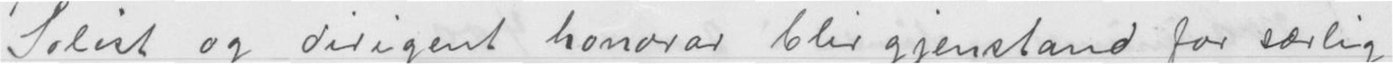Solist og dirigent honorar blir gjenstand for særlig
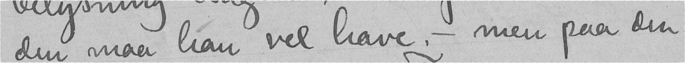den maa han vel have, - men paa den
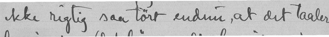ikke rigtig saa tørt endnu, at det taaler
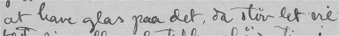at have glas paa det, da støv let vil
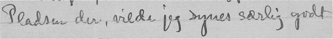Pladsen der, vilde jeg synes særlig godt
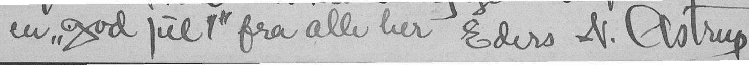en "god jul!" fra alle her Eders N. Astrup
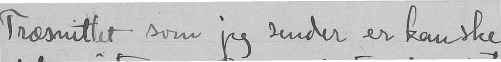Træsnittet som jeg sender er kanske
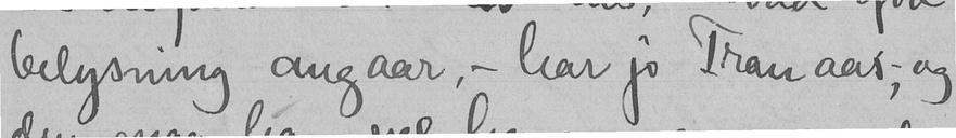belysning angaar, - har jo Tranaas, og
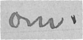om.
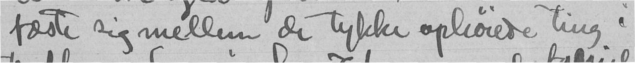fæste sig mellem de tykke ophøiede ting i
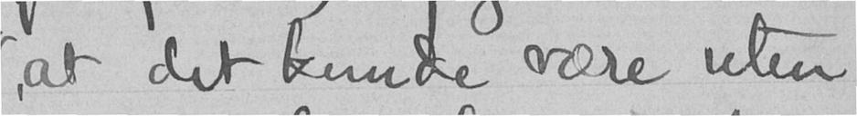, at det kunde være uten
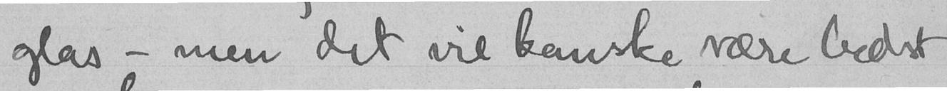glas - men det vil kanske være bedst
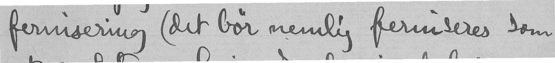fernisering (det bør nemlig ferniseres som
